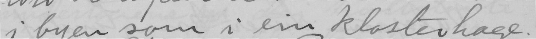i byen som i ein klosterhage.
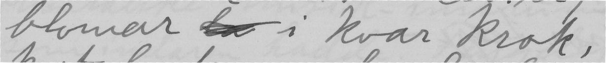blomar  i kvar krok,
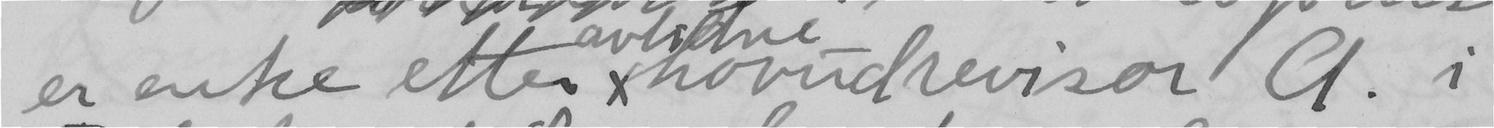er enke etter avlidne hovudrevisor A. i
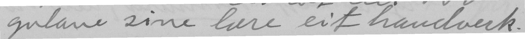gutane sine lære eit handverk.
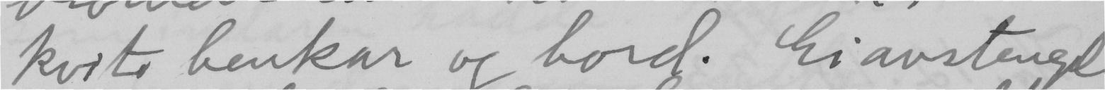kvita benkar og bord. Ei avstengd
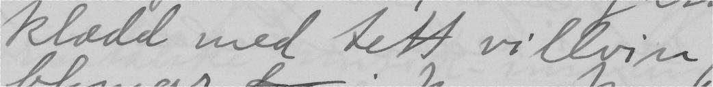klædd med tett villvin,
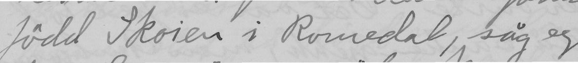född Skoien i Romedal, såg eg
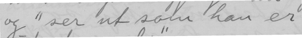og "ser ut som han er
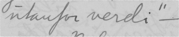utanfor verdi" –
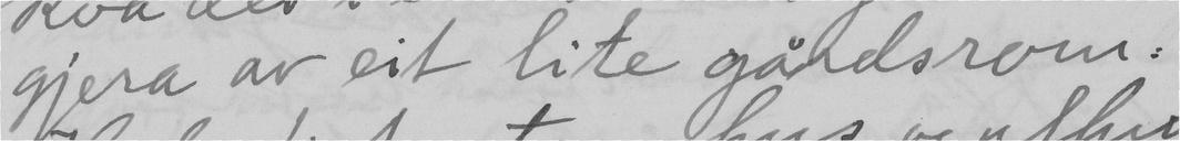gjera av eit lite gårdsrom.
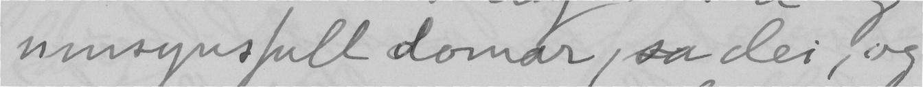umsynsfull domar, sa dei, og
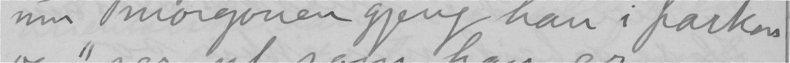um morgonen gjeng han i parkene
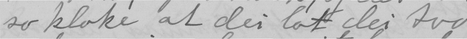so kloke at dei lot dei tvo
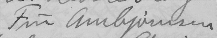Fru Ambjörnsen
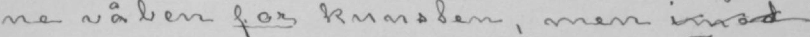ne våben for kunsten, men imod
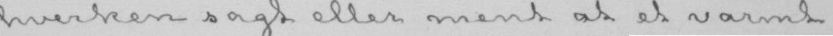hverken sagt eller ment at et varmt
